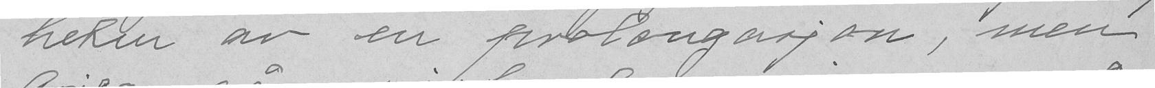heten av en prolongation, men
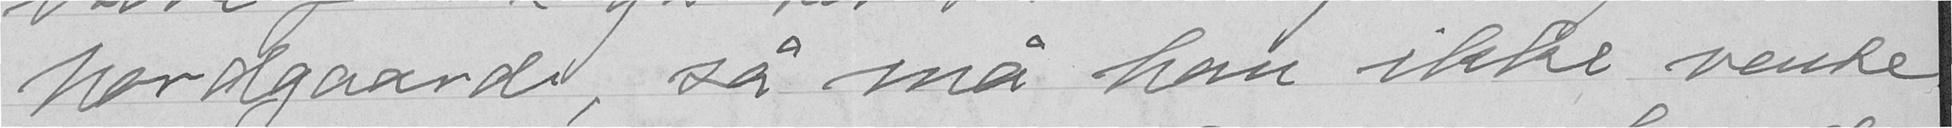Nordgaard, så må han ikke vente
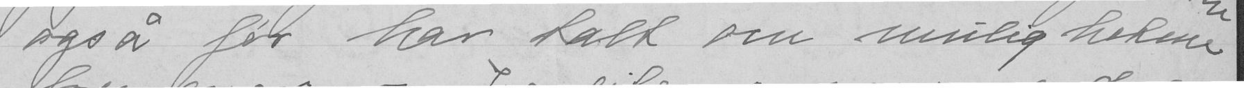også før har talt om mulighetene
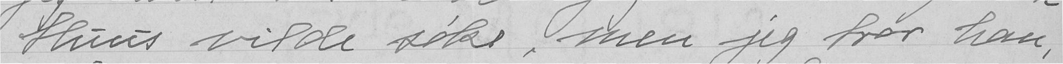Huus vilde søke, men jeg tror han,
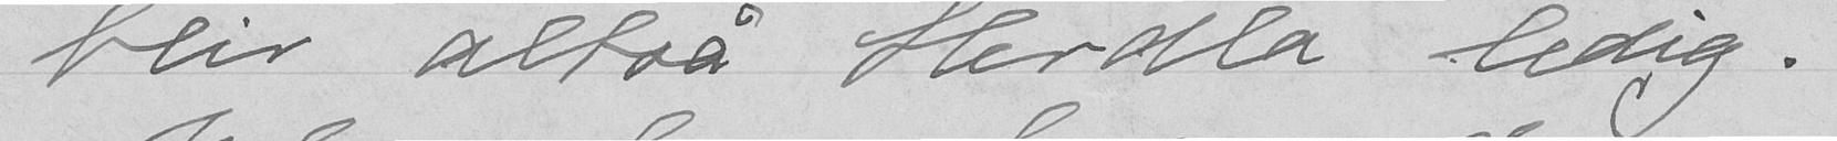blir altså Herdla ledig.
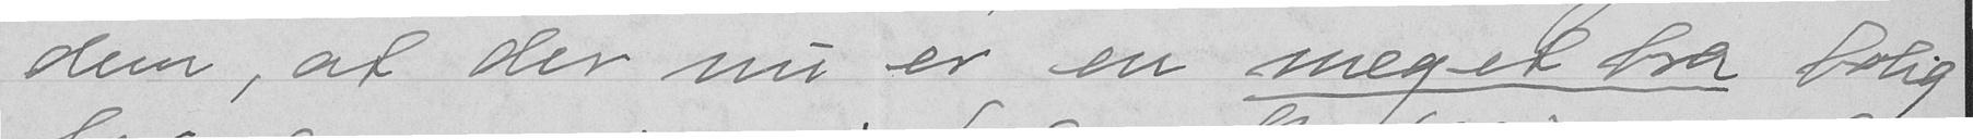dem, at der nu er en meget bra bolig
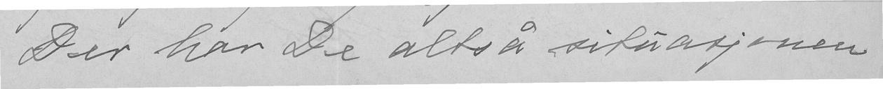Der har De altså situasjonen
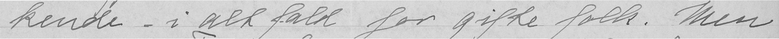kende - i alt fald for gifte folk. Men
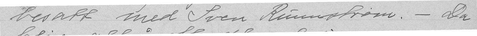besatt med Sven Runnstrøm. - Da
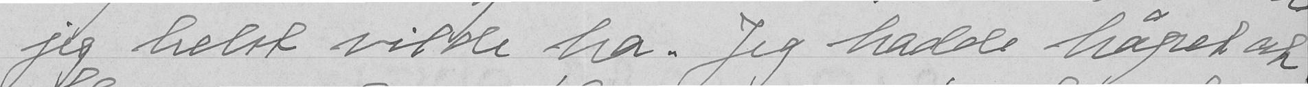jeg helst vilde ha. Jeg hadde håpet at
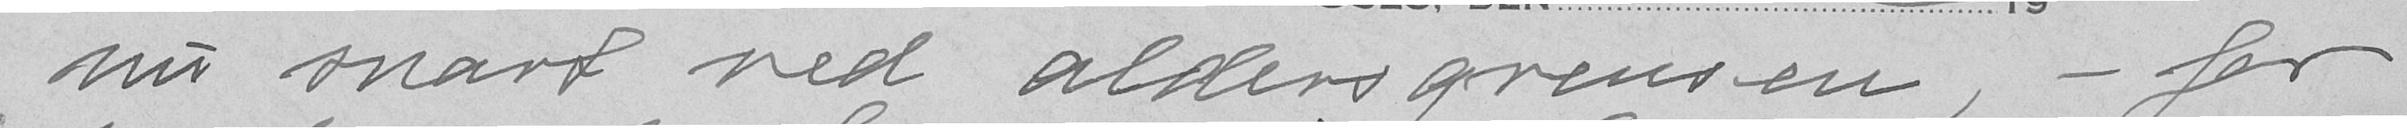nu snart ved aldersgrensen, - for
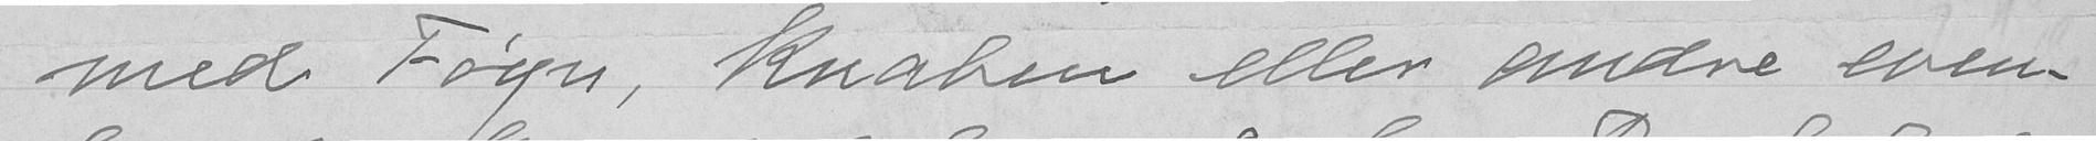med Føyn, Knaben eller andre even-
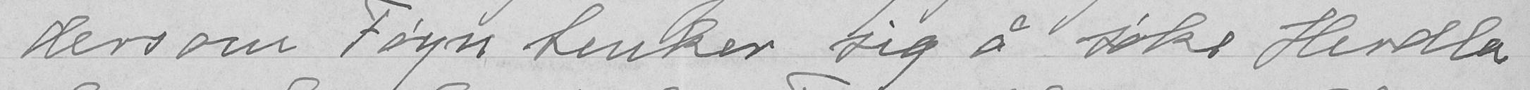dersom Føyn tenker sig å søke Herdla
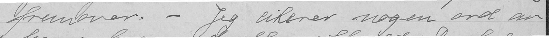fremover. - Jeg citerer nogen ord av
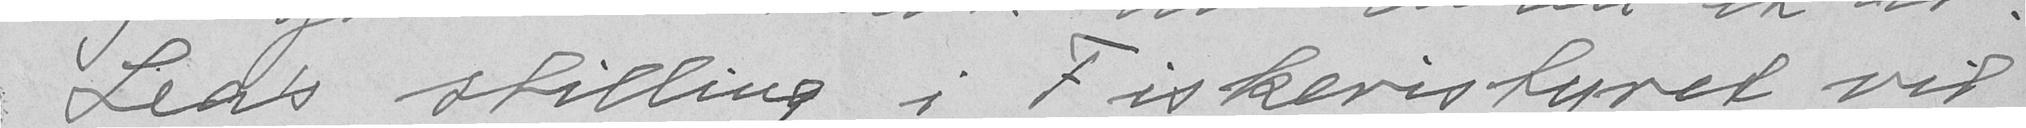Lea's stilling i Fiskeristyret vil
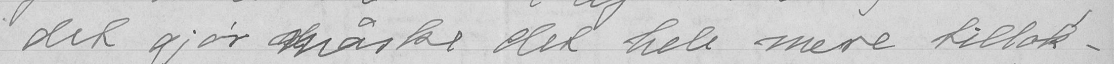det gjør måske det hele mere tillok-
